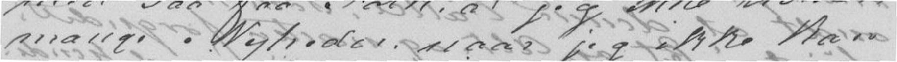mange Nyheder, naar jeg ikke kan
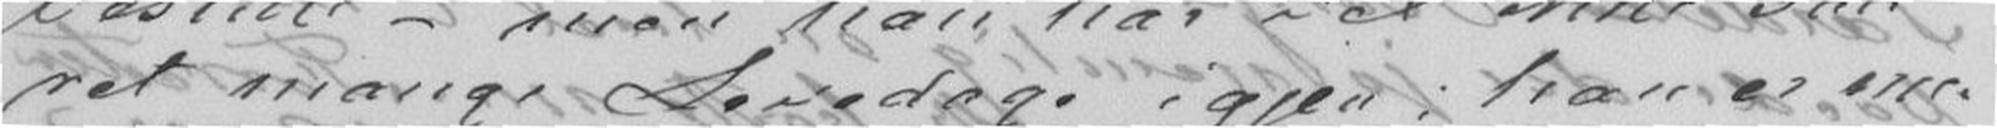ret mange Levedage igjen; han er me-
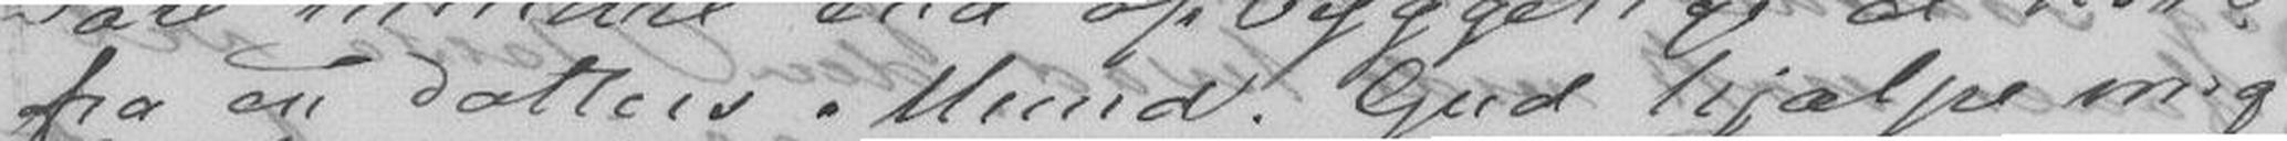fra en Datters Mund. Gud hjælpe mig
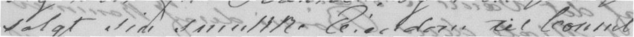solgt sin smukke Eiendom til Consul
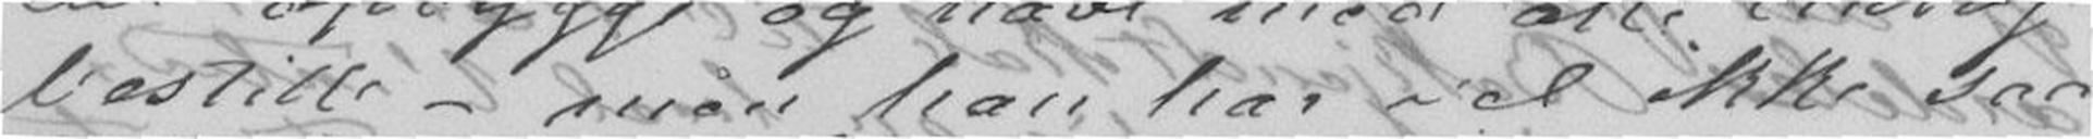bestille - men han har vel ikke saa
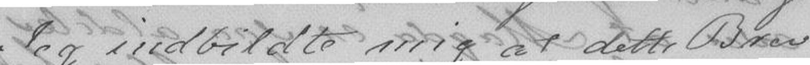Jeg indbildte mig at dette Brev
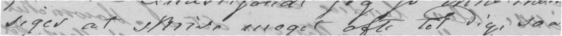siges at skrive meget ofte til Dig, saa
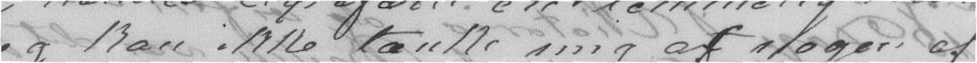jeg kan ikke tænke mig at nogen af
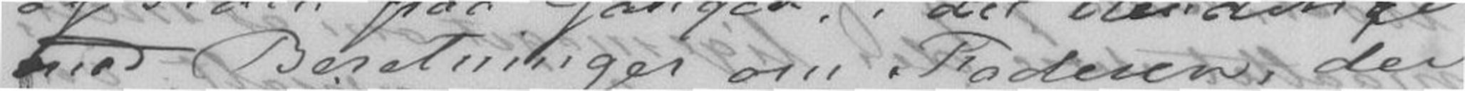med Beretninger om Faderen, der
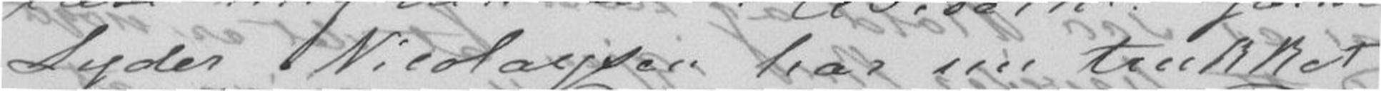Lyder Nicolaysen har nu trukket
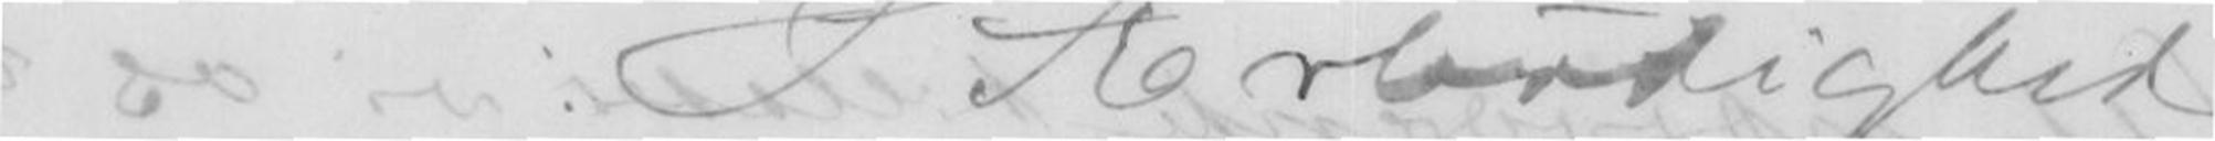I Ærbødighed
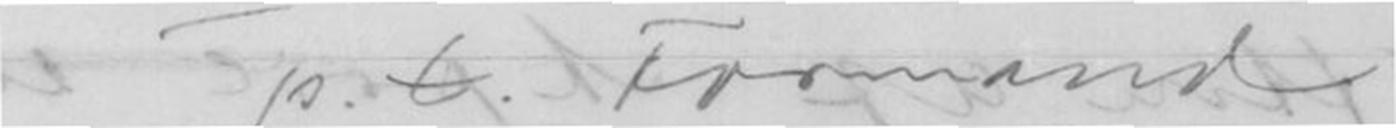p.t. Formand
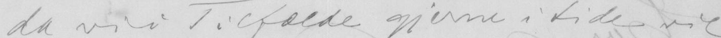da vi i Tilfælde gjerne i tide vil
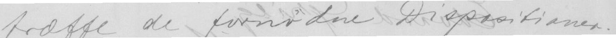træffe de fornødne Dispositioner. -
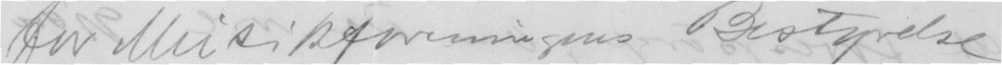for Musikforeningens Bestyrelse
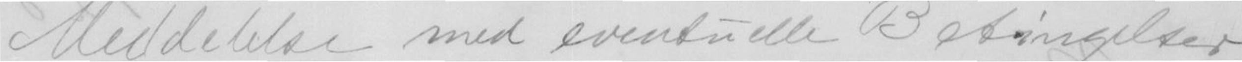Meddelelse med eventuelle Betingelser,
